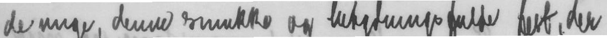de unge, denne smukke og betygningsfulde fest, der
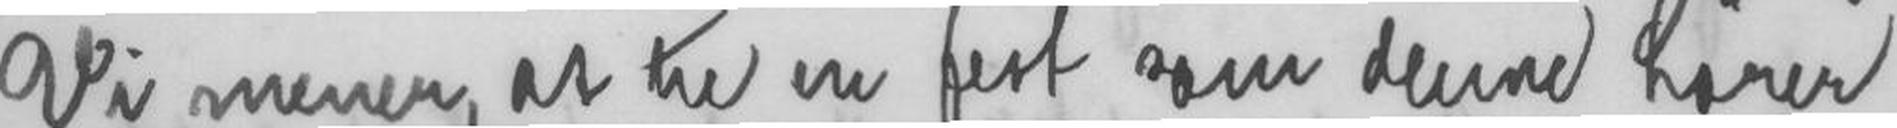Vi mener, at til en fest som denne hører
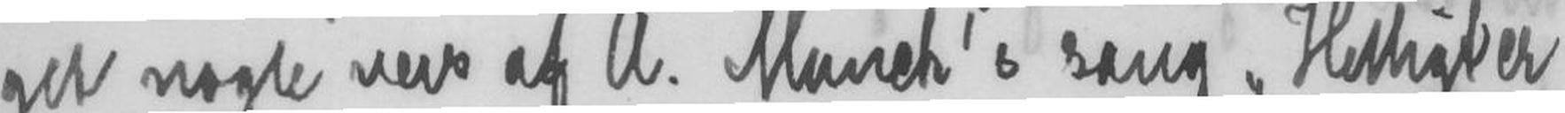get nogle vers af A. Munch's sang Helligt er
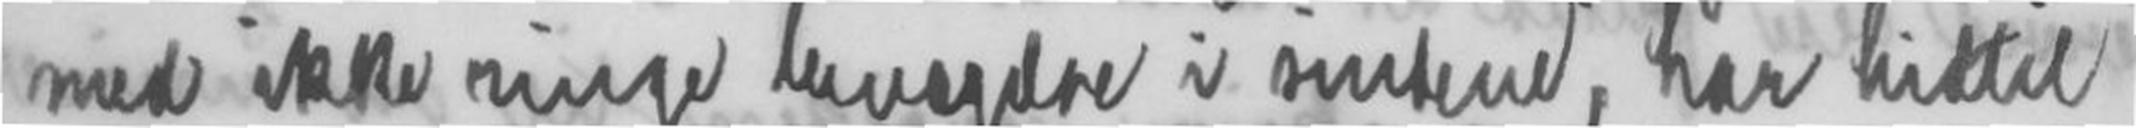med ikke ringe bevegelse i sindene, har hittil
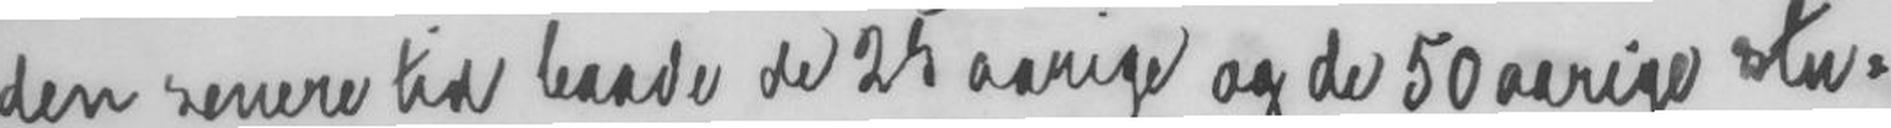den senere tid baade de 25 aarige og de 50 aarige stu-
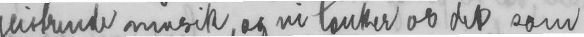geistrende musik, og vi tænker os det som
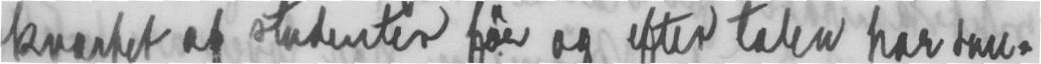kvartet af studenter før og efter talen sun-
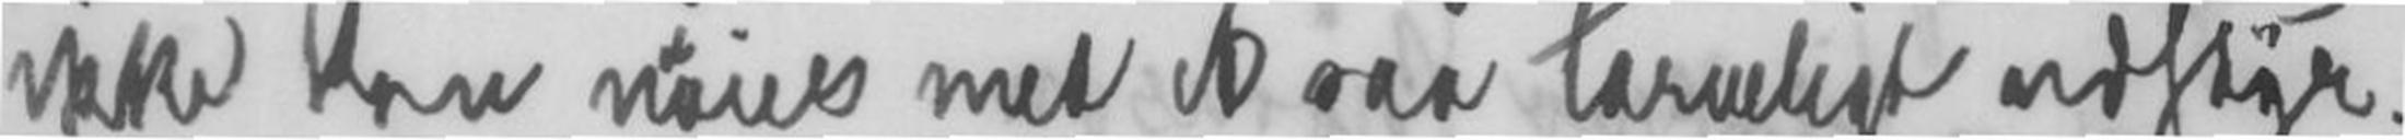ikke kan nøies med et saa tarveligt udstyr.
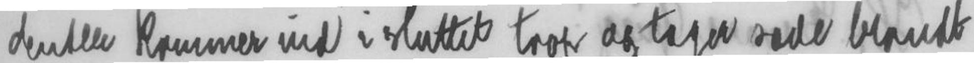denter kommer ind i sluttet trop og tager sæde blandt
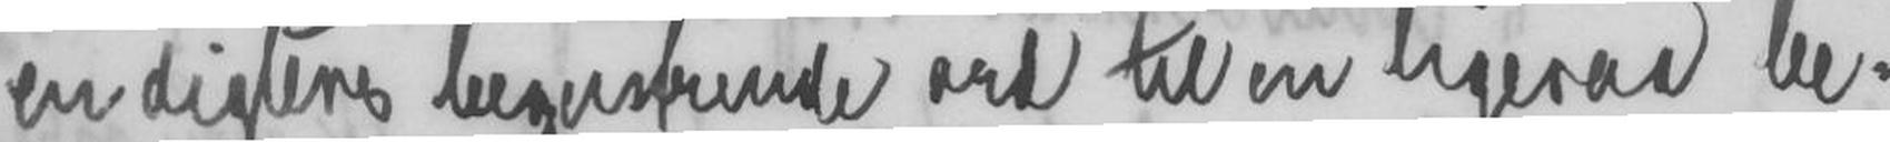en digters begeistrende ord til en ligesaa be-
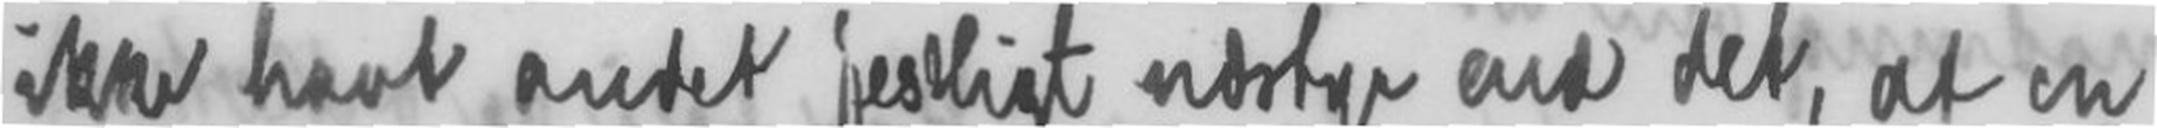ikke havt andet festligt udstyr end det, at en
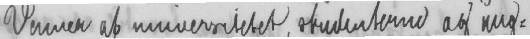Venner af universitetet, studenterne og ung-
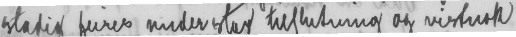stadig feires under stor tilslutning og vistnok
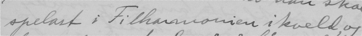spelast i Filharmonien ikveld og
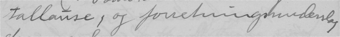tallause, og forretningsunderslag
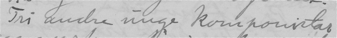Tri andre unge komponistar
